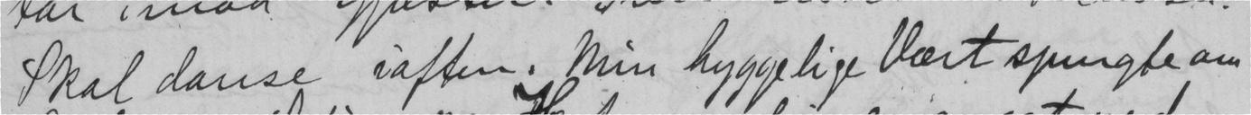Skal danse iaften. Min hyggelige Vært spurgte om
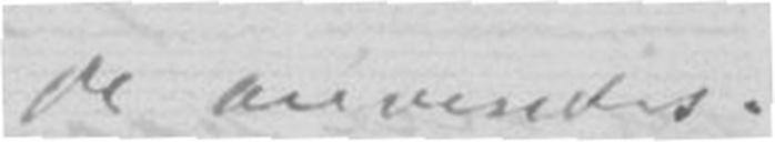e anvendes. -
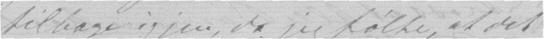tilbage igjen, da jeg følte, at det
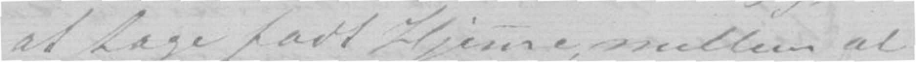at tage fadt Hjeme, mellem al
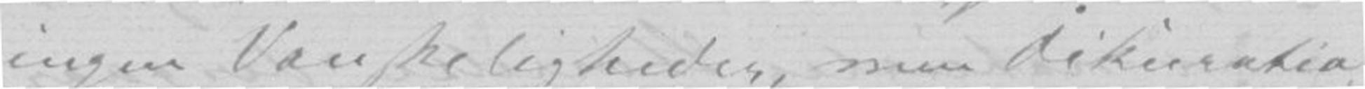ingen Vanskeligheder, men Dikuratia-
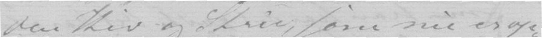den Kiv og Strie, som nu er op-
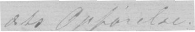dets Opførelse. -
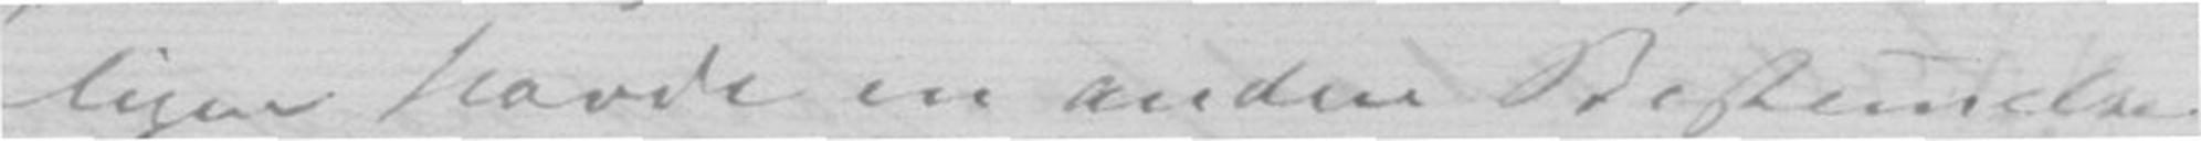ligen havde en anden Bestemelse
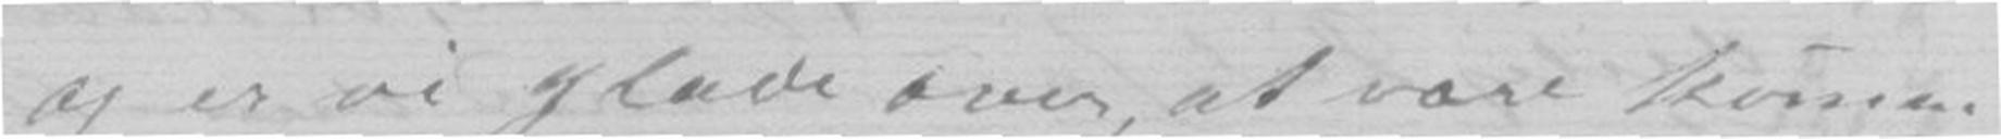og er vi glade over, at være kommen
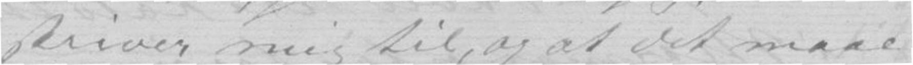skriver mig til, og det maa
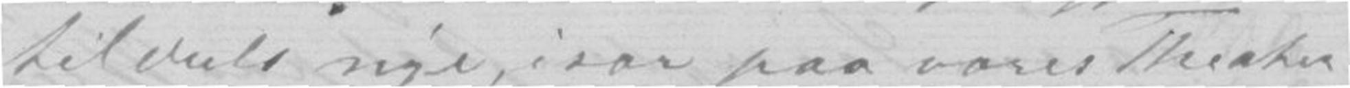til dels nye, især paa vores Theater
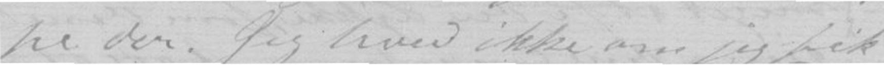pe der. Jeg hved ikke om jeg fik
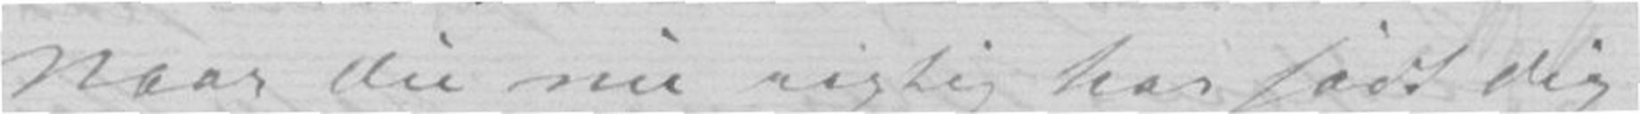Naar du nu rigtig har sadt dig
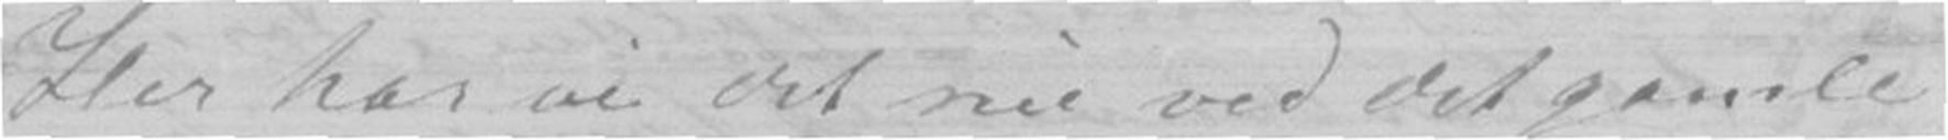Her har vi det nu ved det gamle
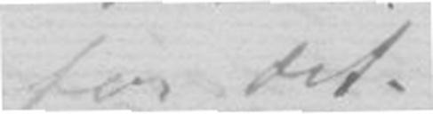for det. -
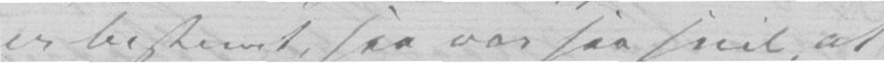er bestemt, saa vær saa snil, at
